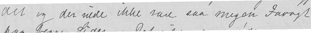det og der vilde ikke være saa megen Foragt
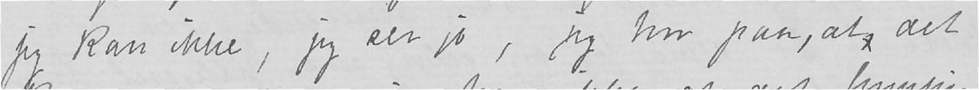jeg kan ikke, jeg ser jo, jeg tror paa, at, det
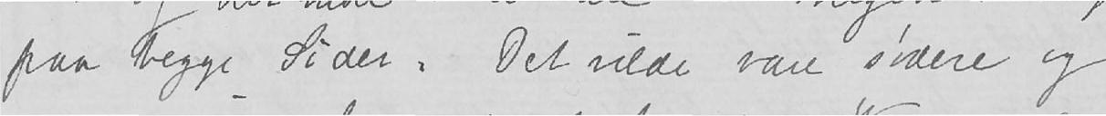paa begge Sider. Det vilde være sødere og
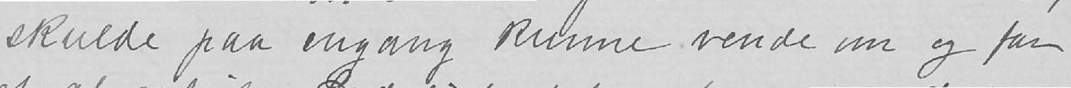skulde paa engang kunne vende om og faa
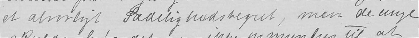et alvorligt Sædelighedsbegreb, men de unge
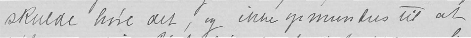skulde høre det, og ikke opmuntres til at
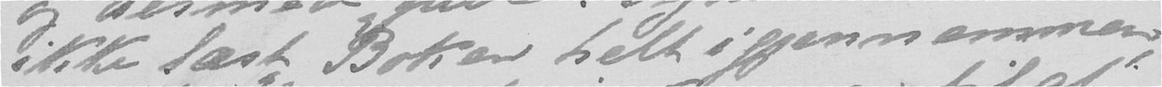ikke læst Boken helt igjennem men,
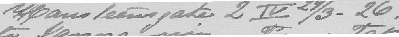Hansteens gate 2 IV 29/3-26.
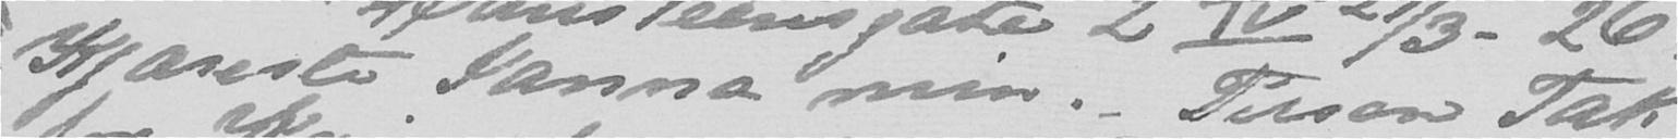Kjæreste Janna min. - Tusen Tak
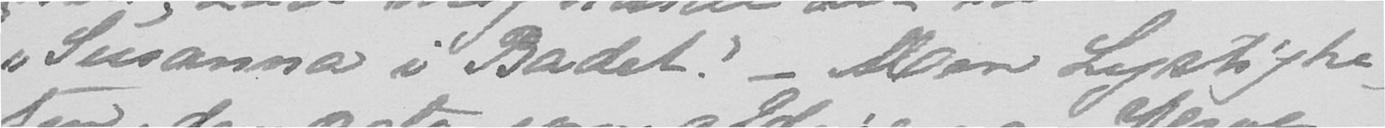"Susanna i Badet." - Men Lystighe-
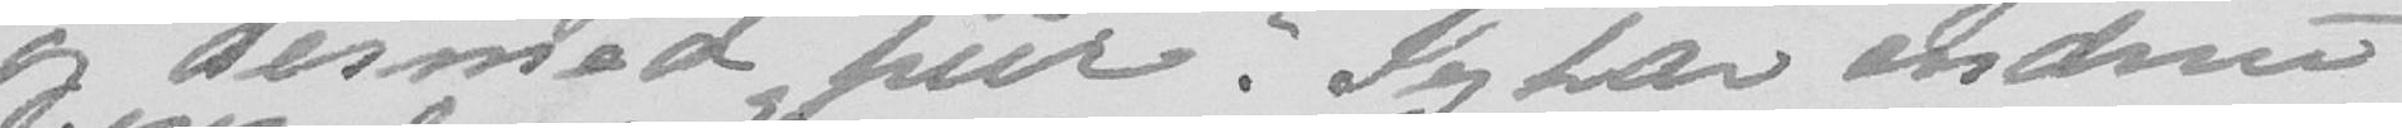dermed pur. Jeg har endnu
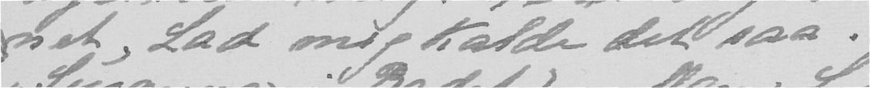net. Lad mig kalde det saa.
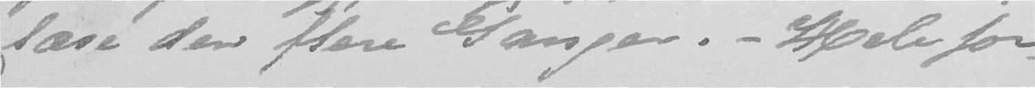læse den flere Ganger. - Hele for-
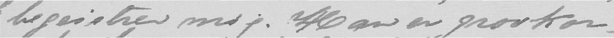begeistrer mig. Han er grovkor-
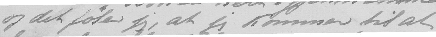og det føler jeg, at jeg kommer til at
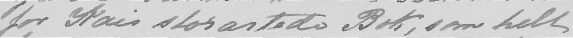for Kais storartede Bok, som helt
